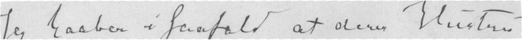Jeg haaber i saafald at Deres Hustru
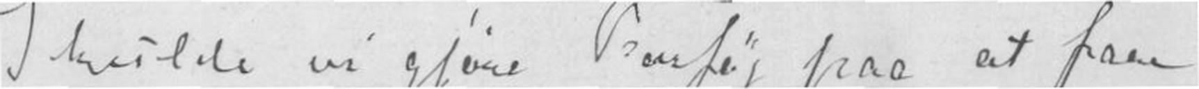Skulde vi gjöre Forsög paa at faae
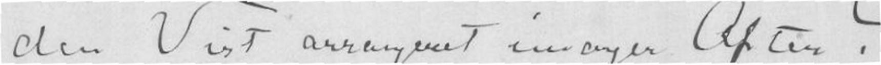den Vist arrangeret imorgen Aften ?
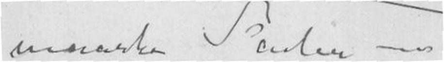maaske Fader
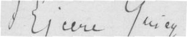Kjære Grieg !
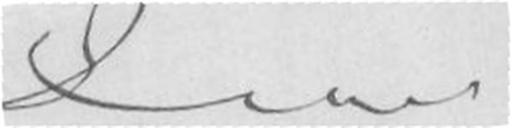Deres
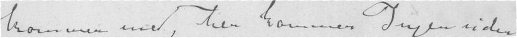kommer med, der kommer Ingen uden
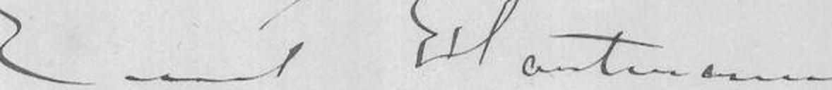Emil Hartmann
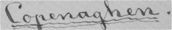Copenaghen.
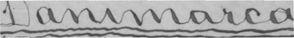Danimarca
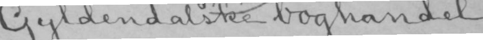Gyldendalske boghandel.
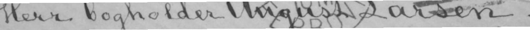Herr bogholder August Larsen
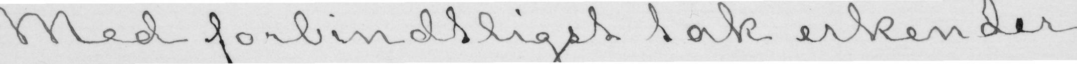Med forbindtligst tak erkender
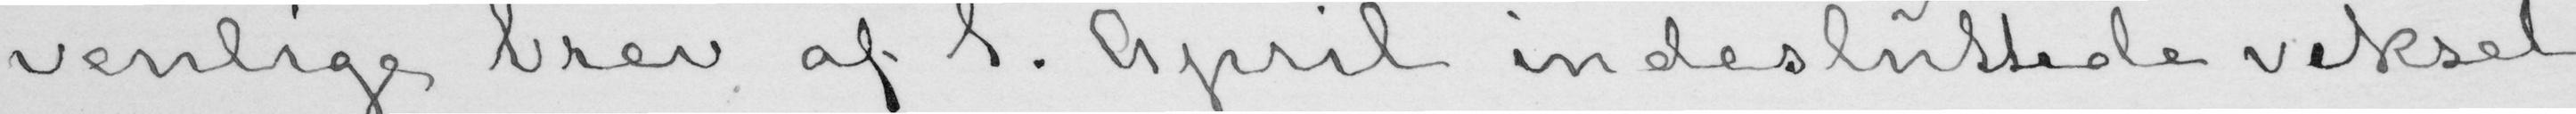venlige brev af 1. April indesluttede veksel
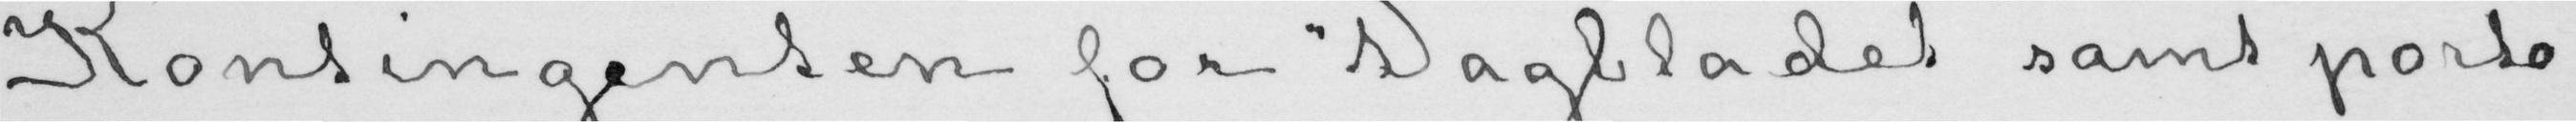Kontingenten for «Dagbladet samt porto
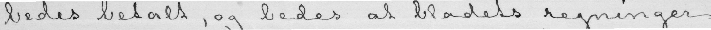bedes betalt, og bedes at bladets regninger
